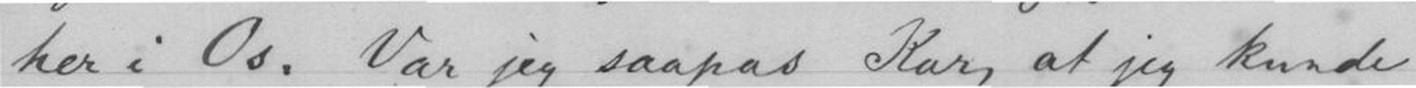her i Os. Var jeg saapas Kar, at jeg kunde
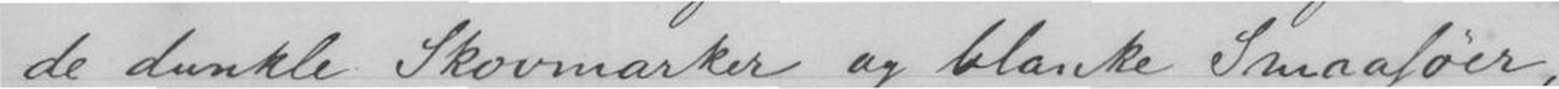de dunkle Skovmarker og blanke Smaasøer,
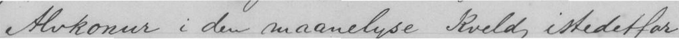Alvkonur i den maanelyse Kveld, istedetfor
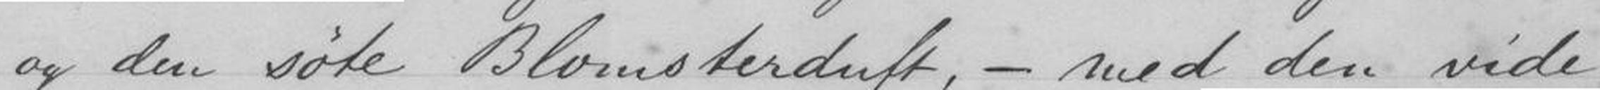og den søde Blomsterduft , - med den vide
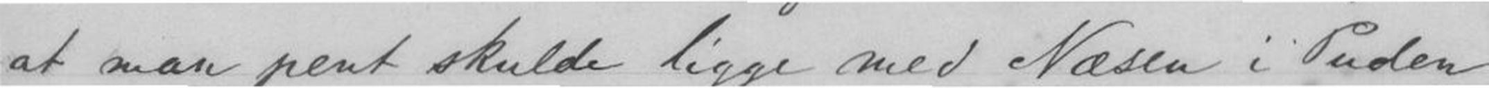at man pent skulde ligge med Næsen i Puden
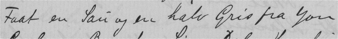Faat en Sau og en halv Gris fra Jon
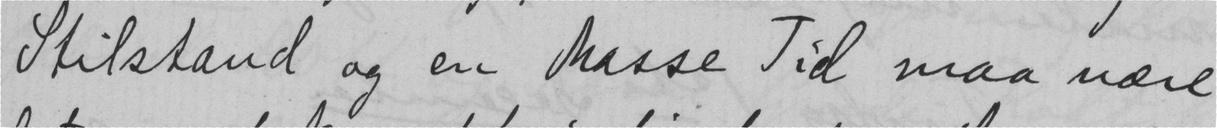Stilstand og en Masse Tid maa være
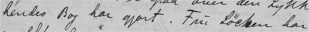hendes Bog har gjort. Fru Løcken har
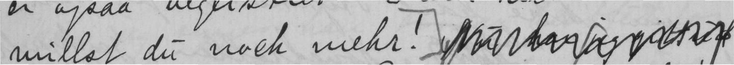willst du noch mehr!
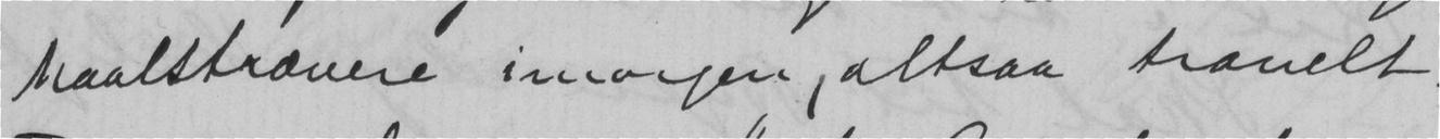maalstravere imorgen, altsaa travelt.
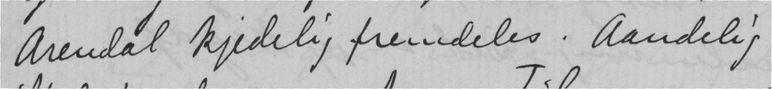Arendal kjedelig fremdeles. Aandelig
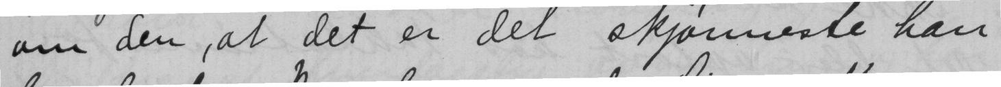om den, at det er det skjønneste han
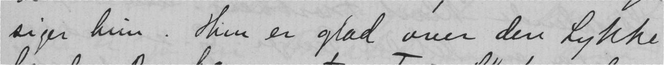siger hun. Hun er glad over den Lykke
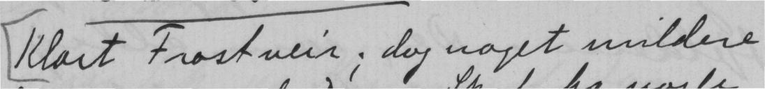Klart Frostvei; dog noget mildere
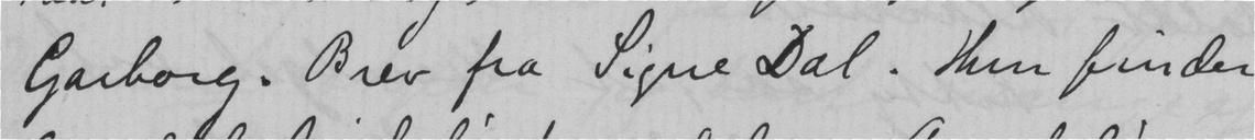Garborg. Brev fra Signe Dal. Hun finder
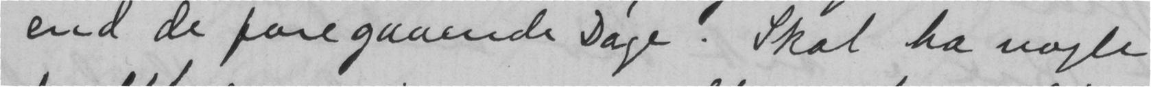end de foregaaende Dage. Skal ha nogle
